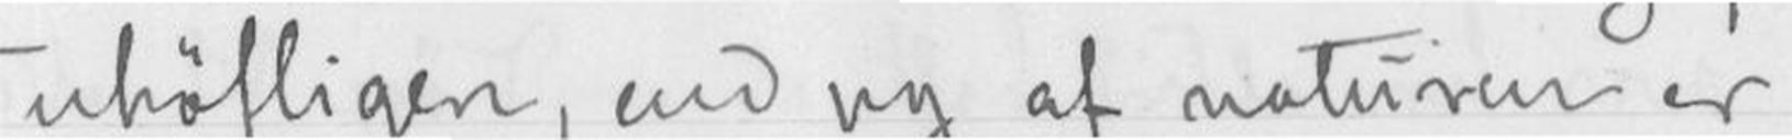uhøfligere, end jeg af naturen er,
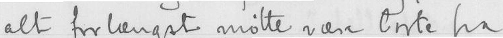alt forlengst måtte være borte fra
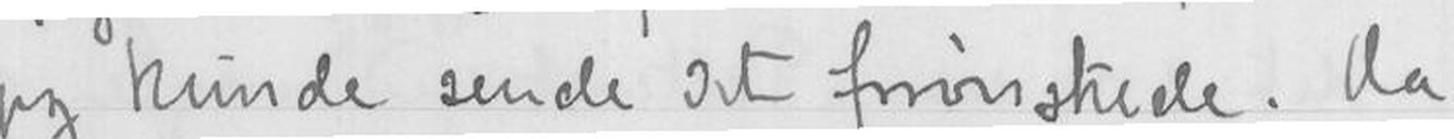jeg kunde sende det forønskede. Da
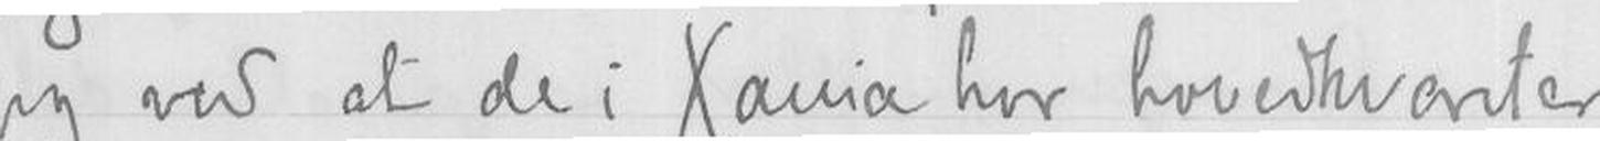jeg ved at de i Xania har hovedkvarter
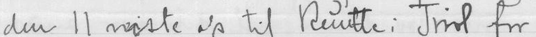den 11 reiste op til Reutte i Tirol for
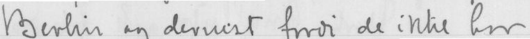Berlin og dernest fordi de ikke har
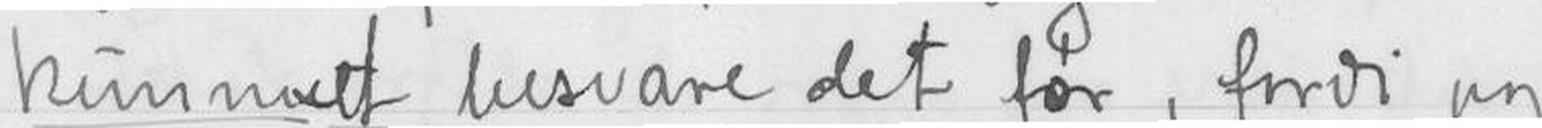kunnet besvare det før, fordi jeg
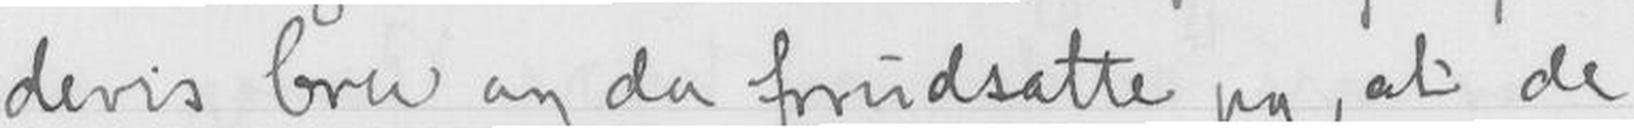deres brev og da forudsatte jeg, at de
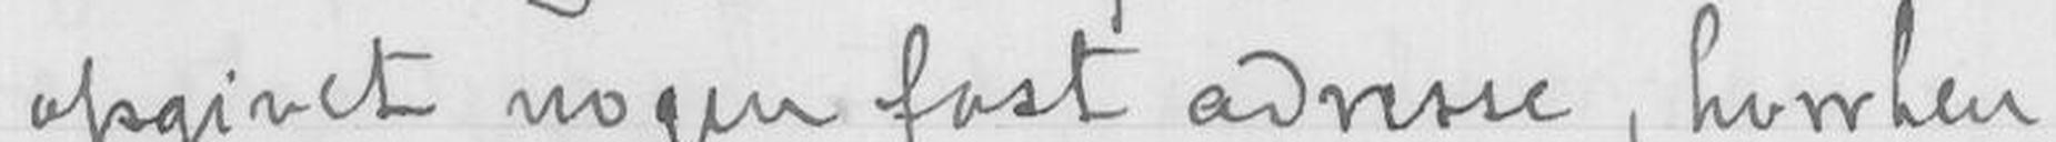opgivet nogen fast adresse, hvorhen
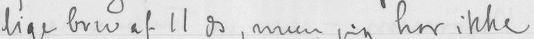lige brev af 11 ds, men jeg har ikke
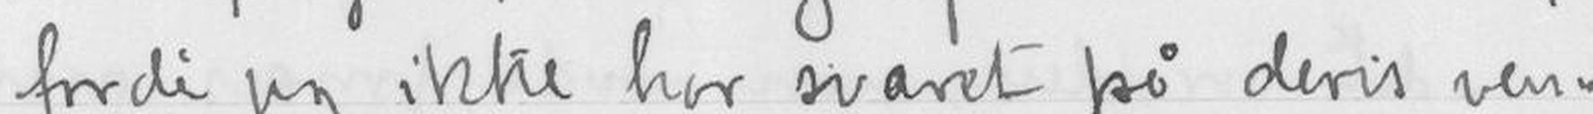fordi jeg ikke har svaret på deres ven-
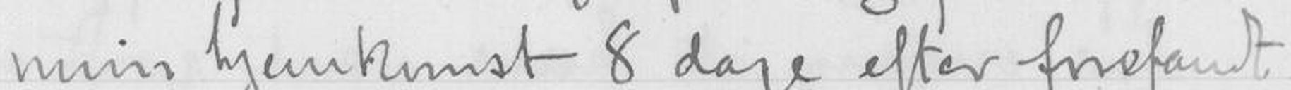min hjemkomst 8 dage efter forefandt
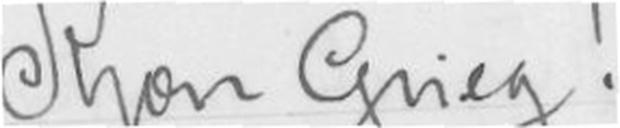Kjære Grieg!
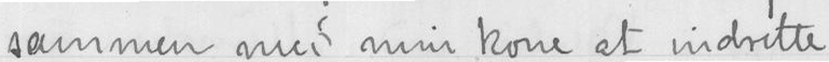sammen med min kone at indrette
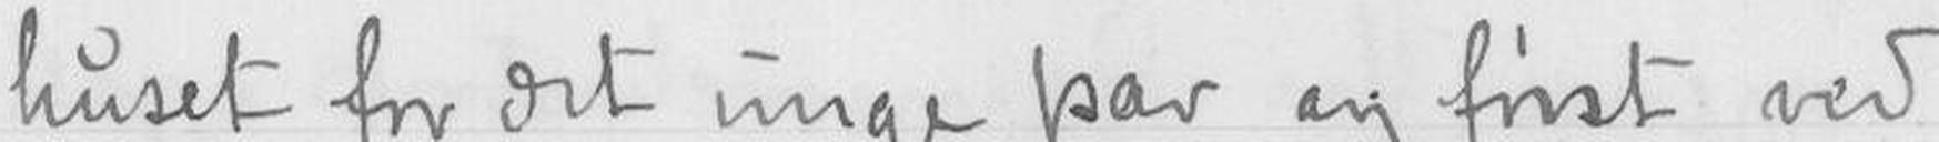huset for sit unge par og først ved
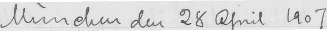München den 28 April 1907
